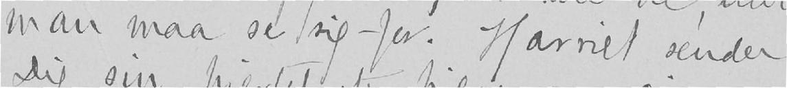man maa se sig for. Harriet sender
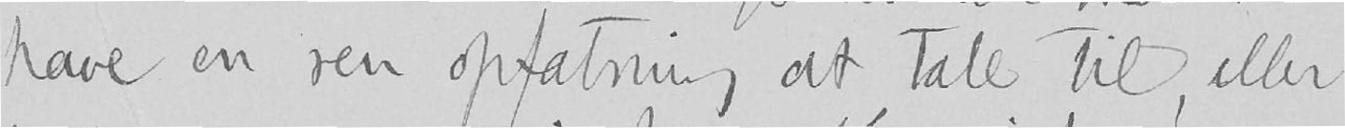have en ren opfatning at tale til, eller
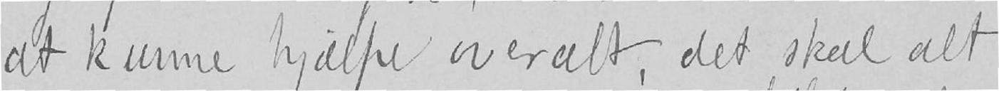at kunne hjælpe overalt, det skal alt
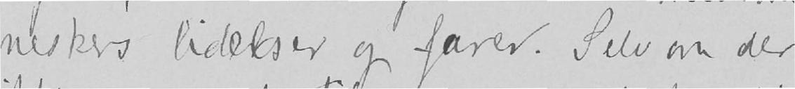neskers lidelser og farer. Selv om der
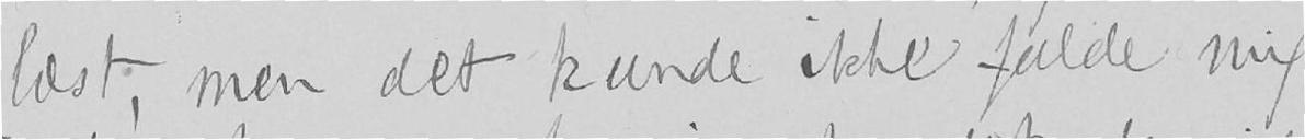læst, men det kunde ikke falde mig
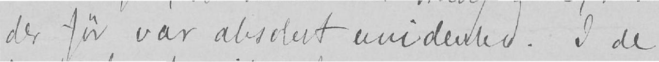der før var absolut uvidenhed. I de
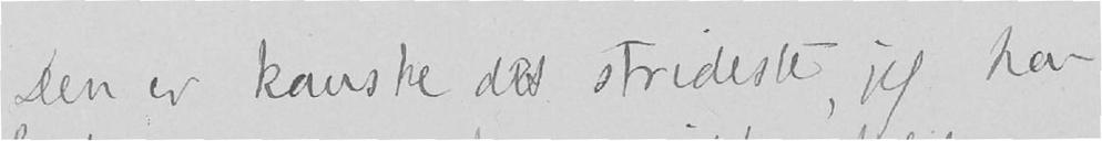Den er kanske det strideste, jeg har
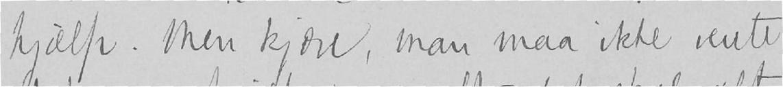hjælp. Men kjære, man maa ikke vente
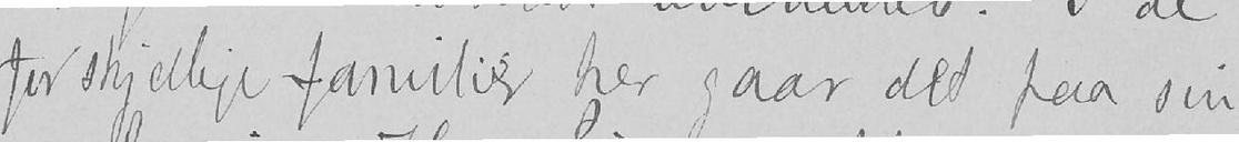forskjellige familier her gaar det paa sin
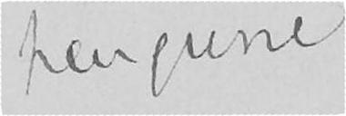hengivne
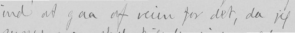ind at gaa af veien for det, da jeg
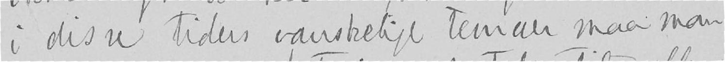i disse tiders vanskelige temaer maa man
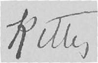Kitty
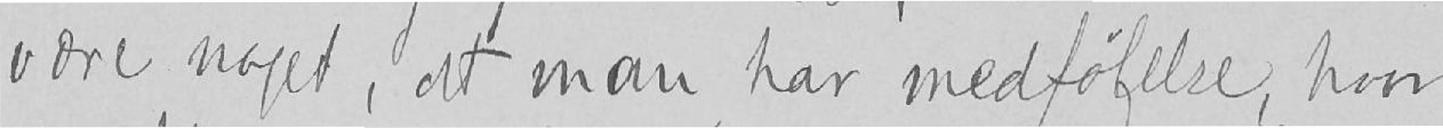være noget, at man har medfølelse, hvor
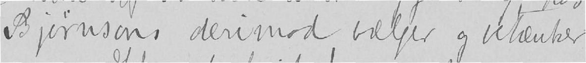Bjørnsons derimod vælger og betænker
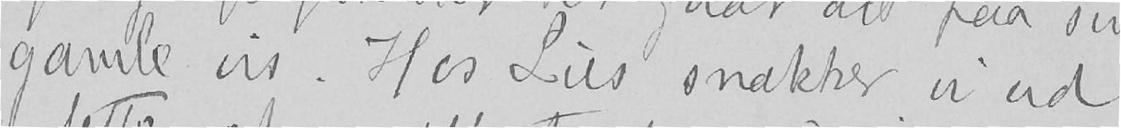gamle vis. Hos Lies snakker vi ud
